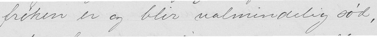frøken er og blir ualmindelig sød,
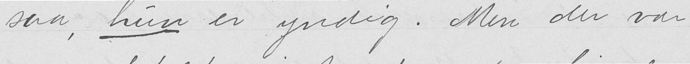saa, hun er yndig. Men der var
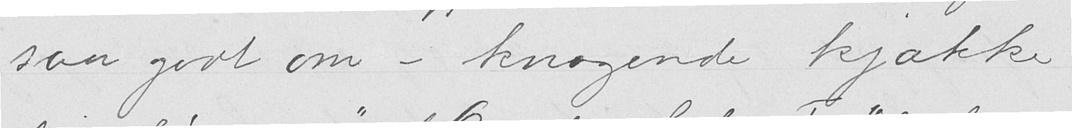saa godt om - knagende kjække
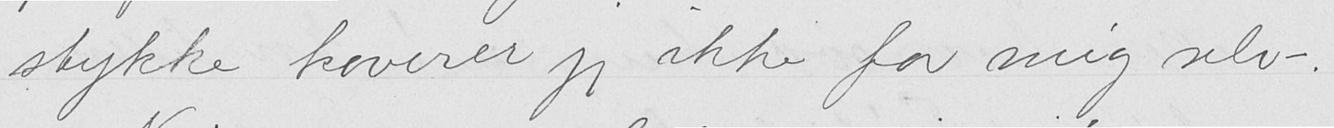stykket hoverer jeg ikke for mig selv -.
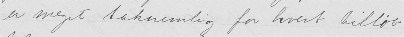er meget taknemlig for hvert tilløb
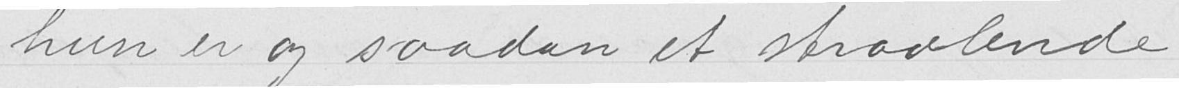hun er og saadan et straalende
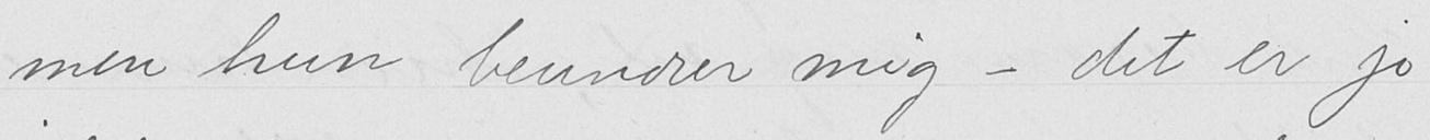men hun beundrer mig - det er jo
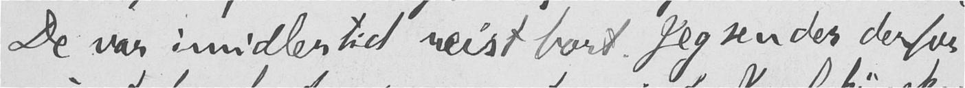De var imidlertid reist bort. Jeg sender derfor
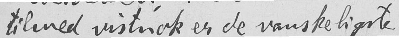tilmed vistnok er de vanskeligste.
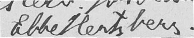Ebbe Hertzberg.
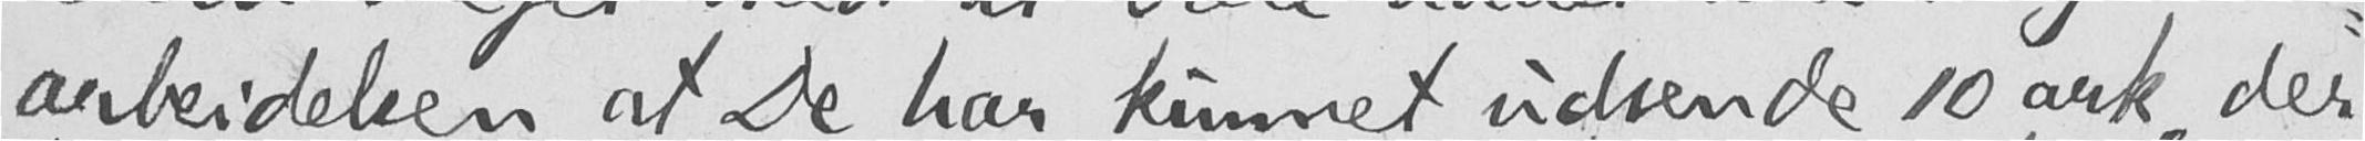arbeidelsen, at De har kunnet udsende 10 ark, der
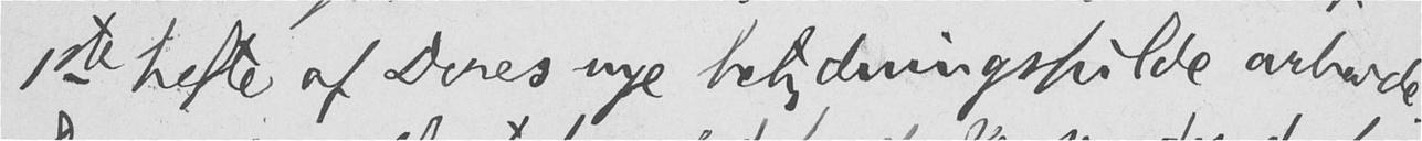1ste hefte af Deres nye betydningsfulde arbeide.
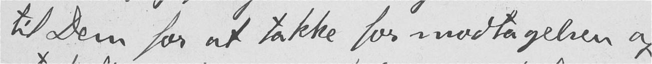til Dem for at takke for modtagelsen af
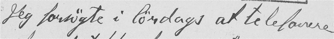Jeg forsøgte i lørdags at telefonere
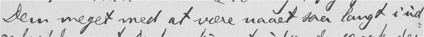Dem meget med at være naaet saa langt i ud-
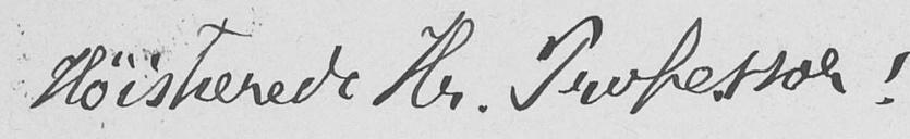Høistærede Hr. Professor!
